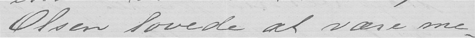Olsen lovede at være me-
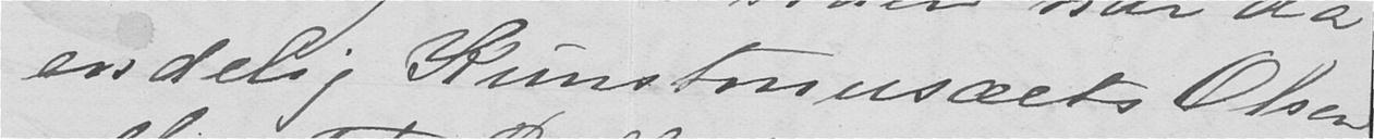endelig Kunstmusæets Olsen
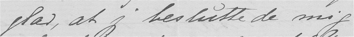glad, at jeg besluttede mig
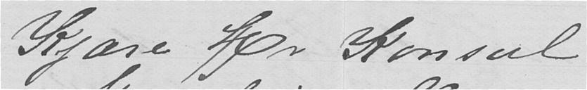Kjære Hr Konsul
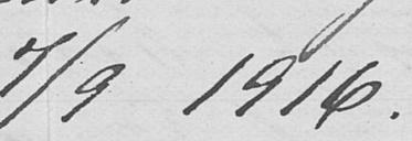7/9 1916.
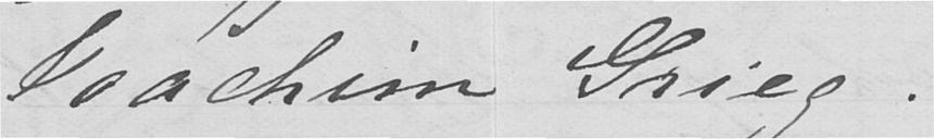Joachim Grieg.
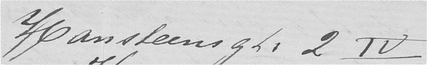Hansteensgt. 2 IV
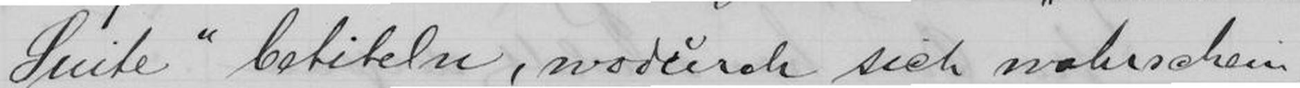Suite betiteln, modurch sich woheschein-
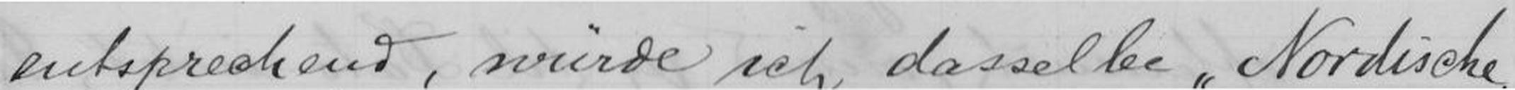entsprechend, würde ich dasselbe Nordische
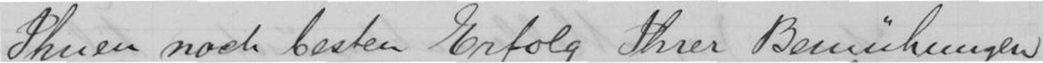Ihnen noch besten Erfolg Ihrer Bemühungen
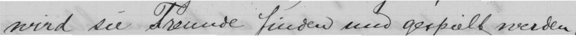wird sie Freunde finden und gespielt werden,
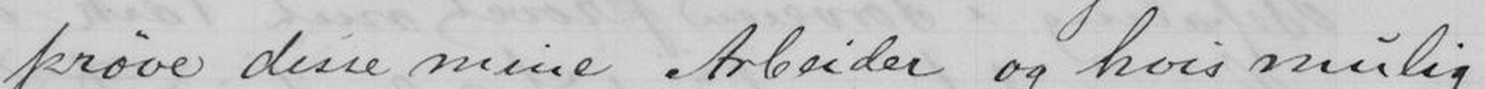prøve disse mine Arbeider og hvis mulig
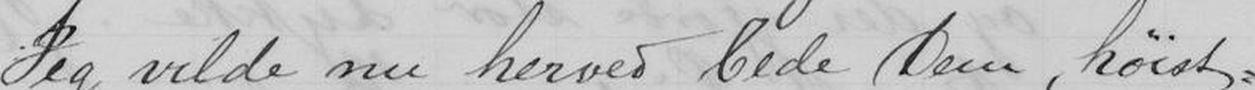Jeg vilde nu herved bede Dem, høist-
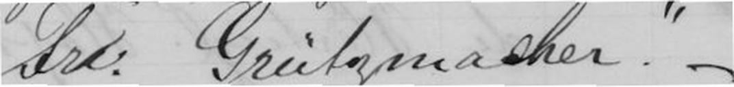Fr. Grützmacher". -
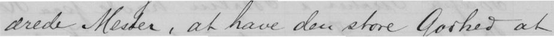ærede Mester, at have den store Godhed at
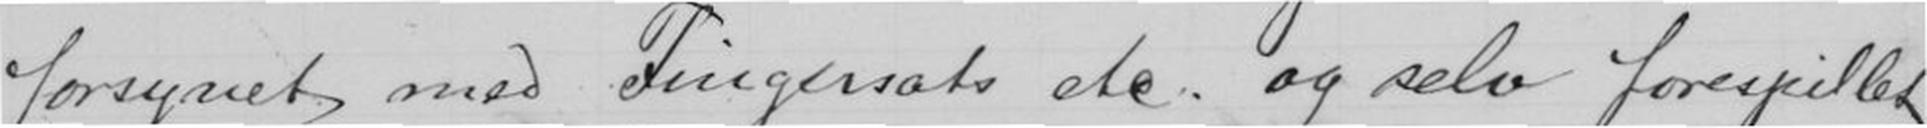forsynet med Fingersats etc. og selv forespillet
